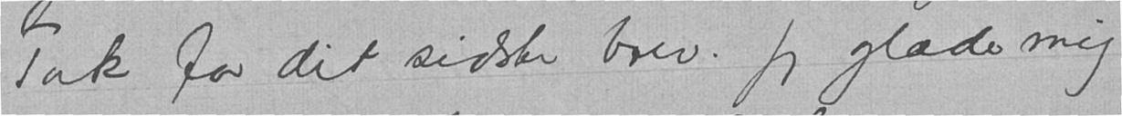Tak for dit sidste brev. Jeg glæder mig
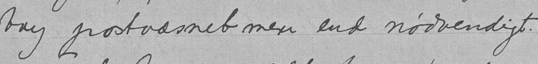bry postvæsnet mere end nødvendigt.
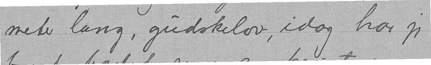meter lang, gudskelov, idag har jeg
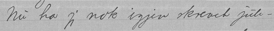Nu har jeg nok igjen skrevet jule-
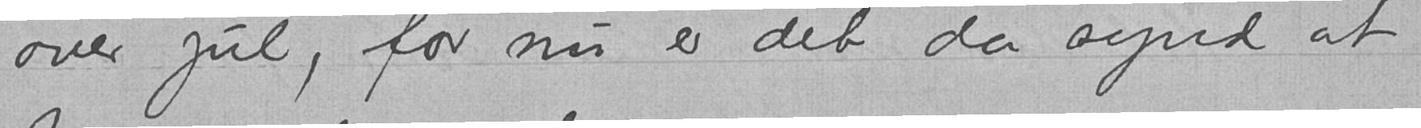over jul, for nu er det da synd at
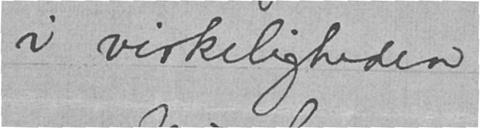i virkeligheden.
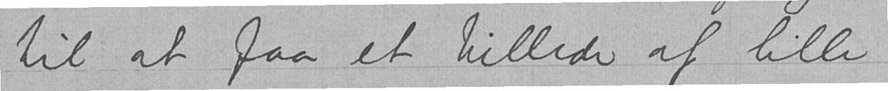til at faa et billede af lille
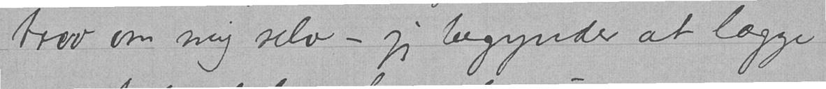brev om mig selv - jeg begynder at lægge
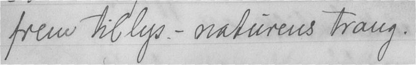frem til lys - naturens trang.
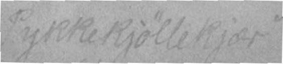"Tykkekjøllekjær"
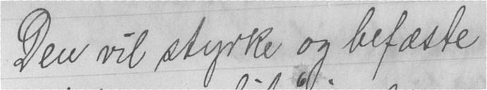Den vil styrke og befæste
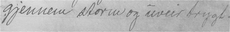gjennem storm og uveir trygt.
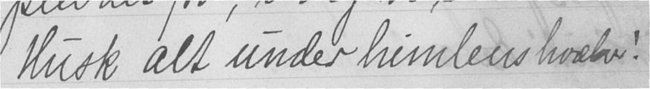Husk alt under himlens hvælv!
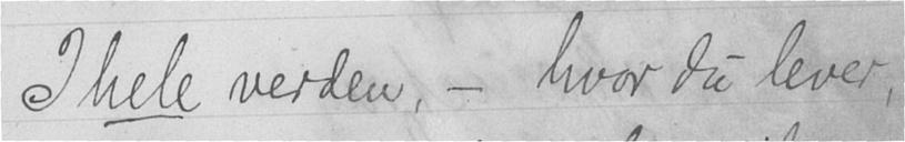I hele verden, - hvor du lever,
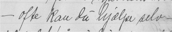- ofte kan du hjælpe selv -
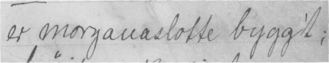er morganaslotte bygg't;
